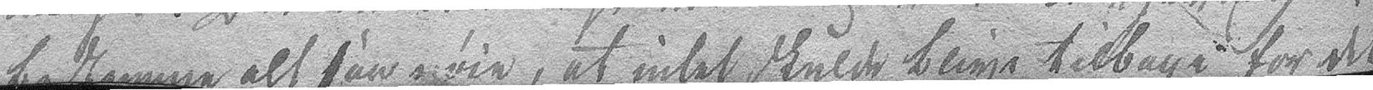bestemme alt saa nøie, at intet skulde blive tilbage for det
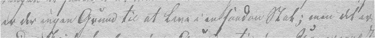er der ingen Grund til at leve i en saadan Stat; men det er
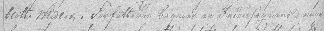blotte Midler. Forfatteren begaaer en Inconseqvens, naaer
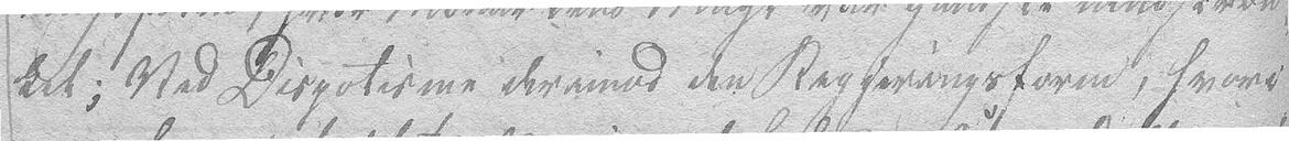ket; Ved Despotisme derimod den Regjeringsform, hvori
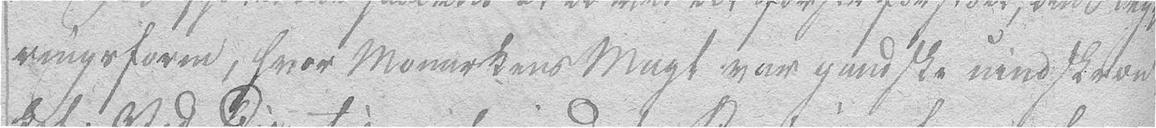ringsform, hvor Monarkens Magt var gandske uindskræn-
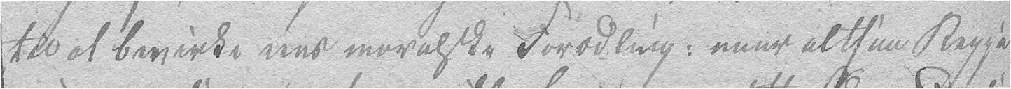til at bevirke eens moralske Forædling: naar altsaa Regje-
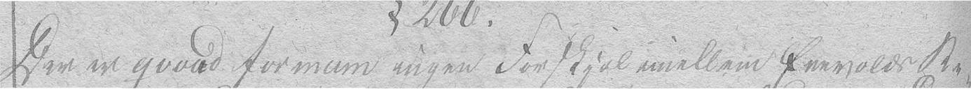Der en qvoud formam ingen Forskjel mellem Enevolds Re-
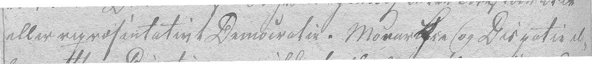eller repræsentativt Democratie. Monarchie og Despotie el-
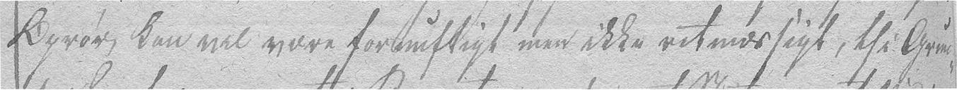Oprør kan vel være fornuftigt men ikke retmæssigt, thi Grun-
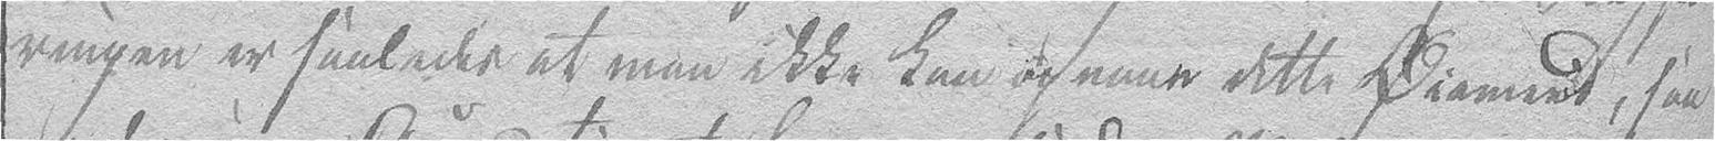ringen er saaledes at man ikke kan opnaae dette Øiemed, saa
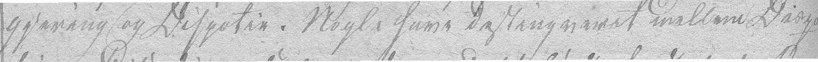gjering og Despotie. Nogle have distingveret mellem Despo-
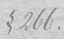§ 266.
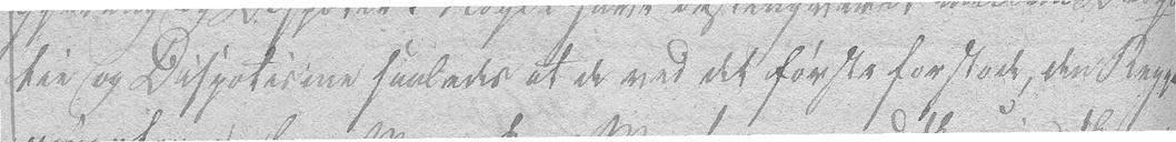tie og Despotisme saaledes at de ved det første forstod, den Regje-
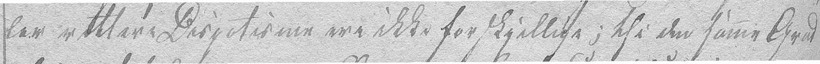ler rettere Despotisme ere ikke forskjellige; thi den samme Grad
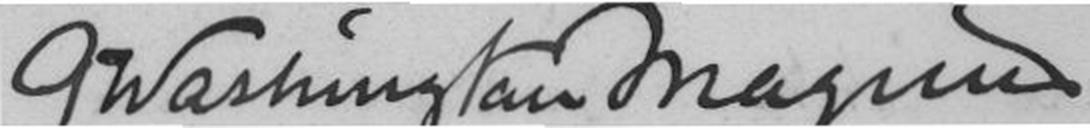G Washington Magnus
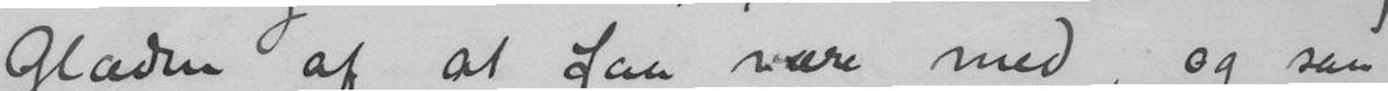Glæden af at faa vare med, og saa
